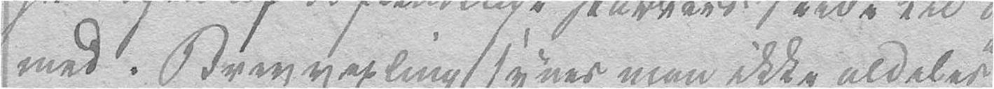med. Brevvexling synes man ikke aldeles
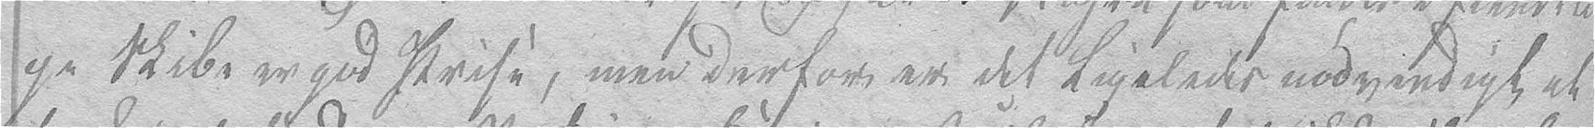ge Skibe er god Prise, men derfor er det ligeledes nødvendigt at
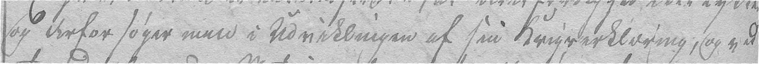og derfor søger man i Udviklingen af sin Krigserklæring, og ved
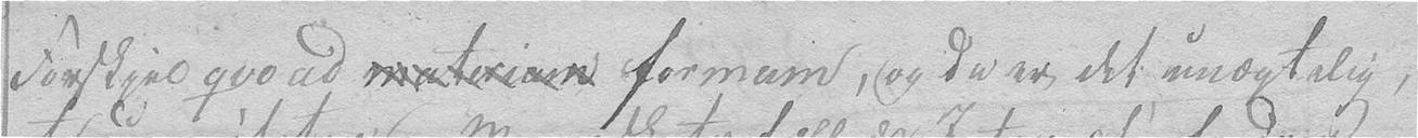Forskjel qvoad materiam formam, og da er det unægtelig,
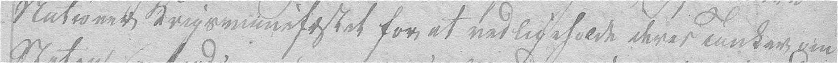Nationer Krigsmanifæstet for at vedligeholde deres Tanker om
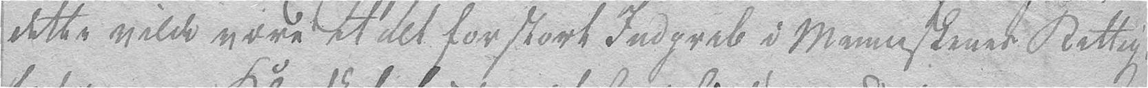dette vilde være et alt for stort Indgreb i Menneskenes Rettig-
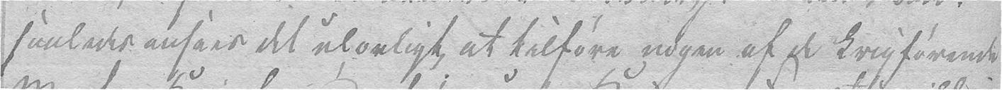saaledes ansees det ulovligt at tilføre nogen af de krigførende
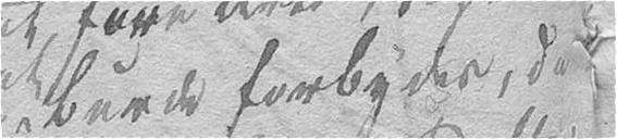burde forbydes, da
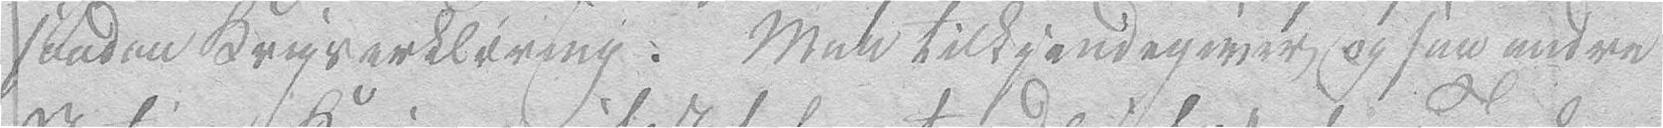saadan Krigserklæring. Man tilkjendegiver ogsaa andre
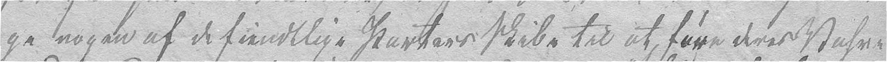ge nogen af de fiendtlige Parters Skibe til at føre deres Vahre
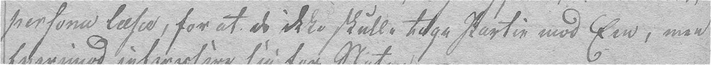persona læsæ, for at de ikke skulle tage Partie mod Een, men
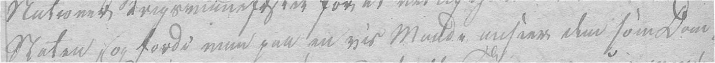Staten, og fordi man paa en vis Maade anseer dem som Dom-
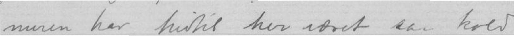meren har hidtil her været saa kold og
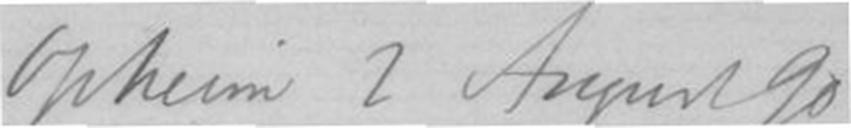Opheim 2 August 90
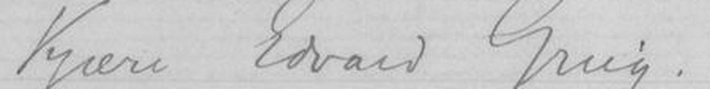Kjære Edvard Grieg.
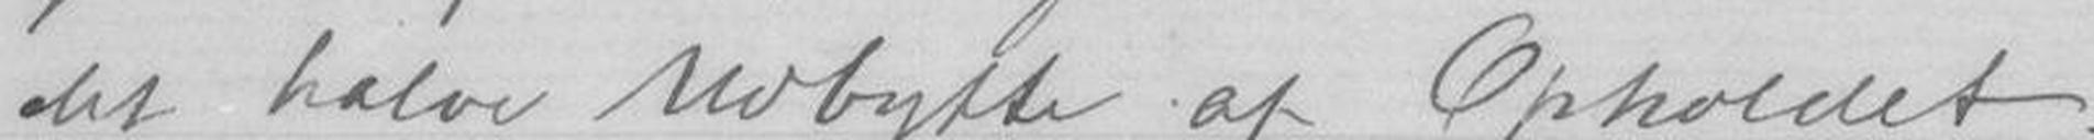det halve Udbytte af Opholdet.
